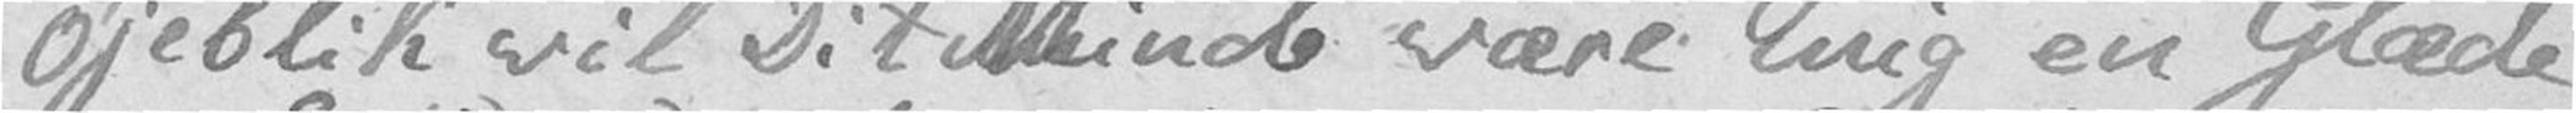Øjeblik vil Dit Minde være mig en Glæde
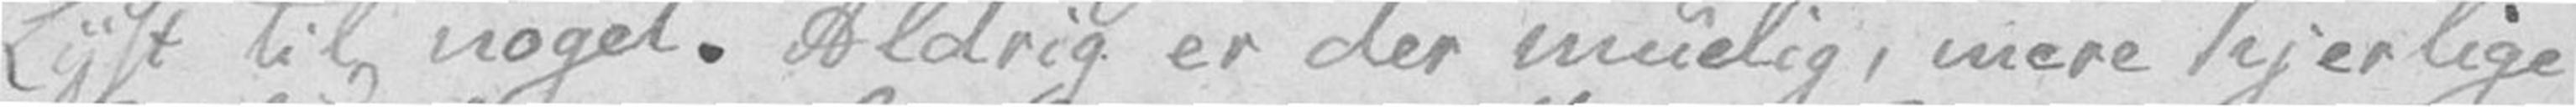Lyst til noget. Aldrig er der muelig, mere kjerlige
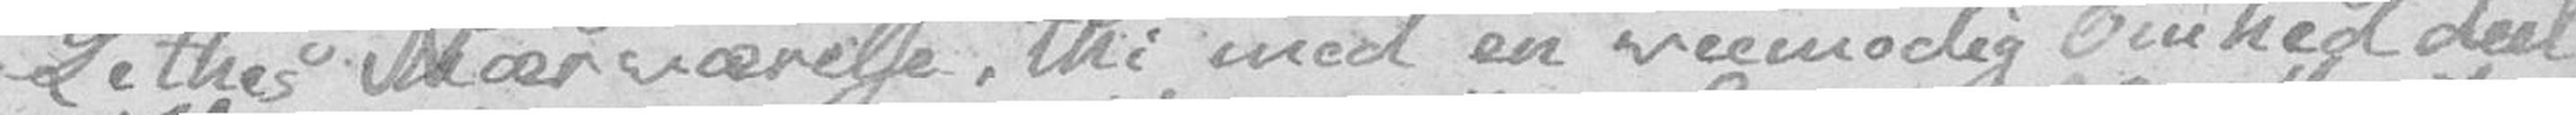Lethes Nærværelse, thi med en veemodig Ømhed deel
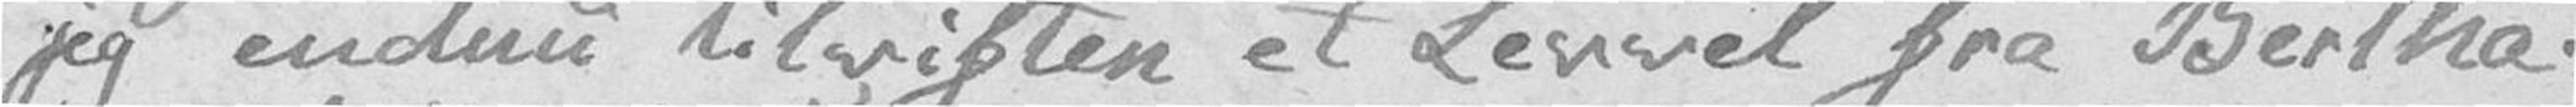jeg endnu tilviften et Levvel fra Bertha!
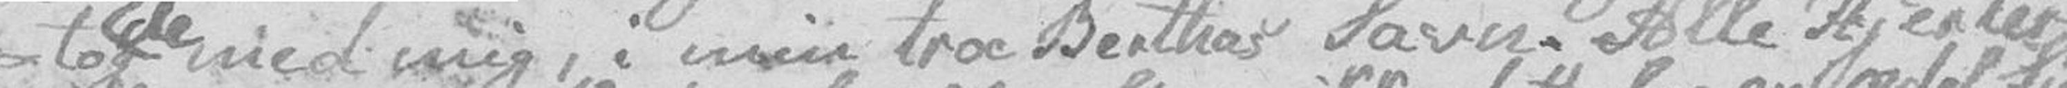-tog de med mig, i min troe Berthas Savn. Alle Hjerter
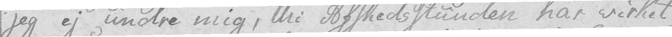jeg ej undre mig, thi Afskedsstunden har virket
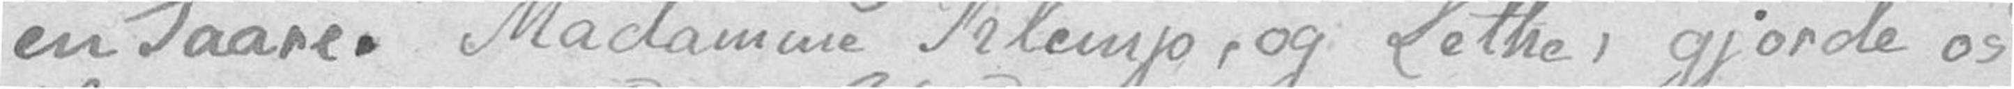en Taare. Madamme Klemp, og Lethe, gjorde os
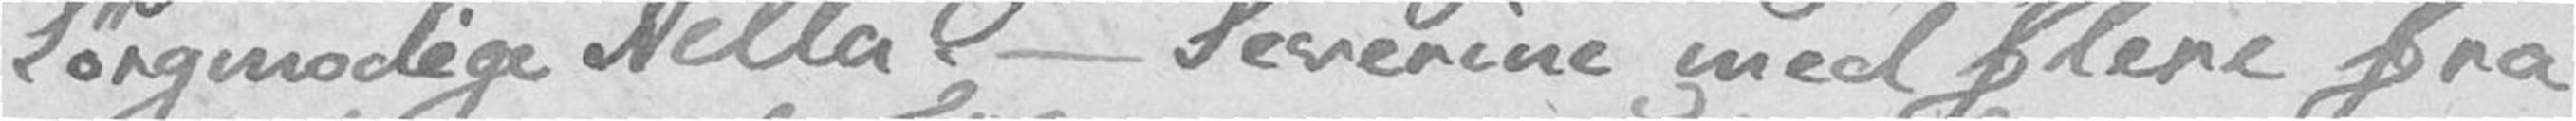Sørgmodige Nella. – Severine med flere fra
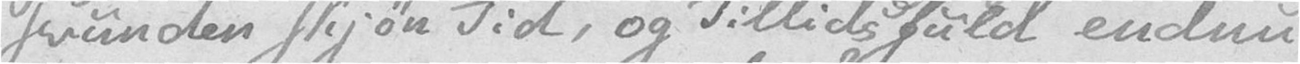svunden skjøn Tid, og Tillidsfuld endnu
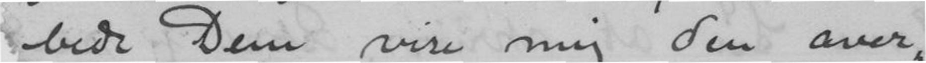bede Dem vise mig den over-
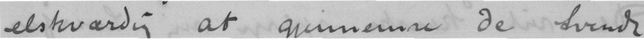elskværdig at gjennemse de tvende
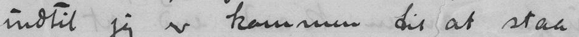indtil jeg er kommen til at staa
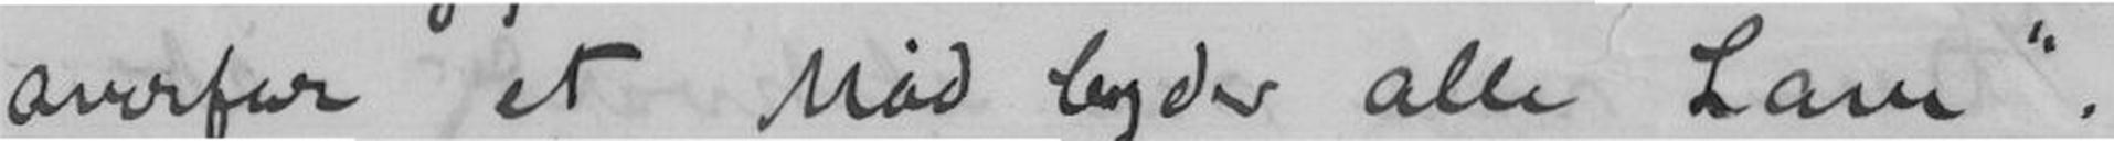overfor et Måd byder alle Lam.
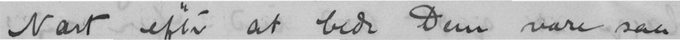Næst efterat bede Dem vare saa
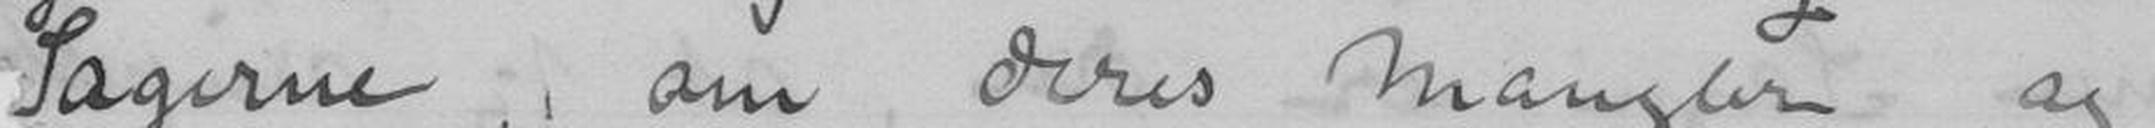Sagerne, om Deres Mangler og
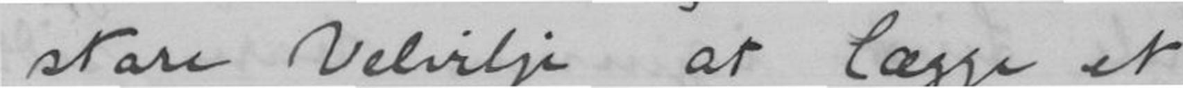store Velvilje at lægge et
